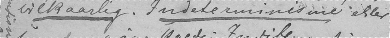vilkaarlig. Indeterminisme eller
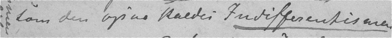som den ogsaa kaldes Indifferentismen
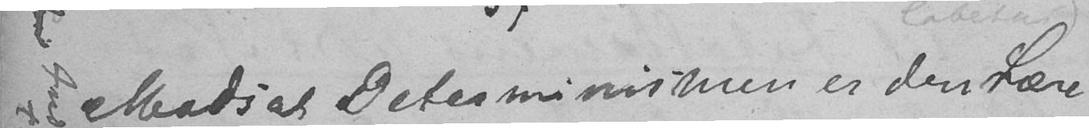Modsat Determinismen er den Lære
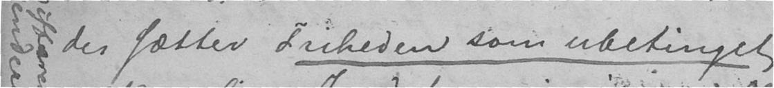der sætter Friheden som ubetinget
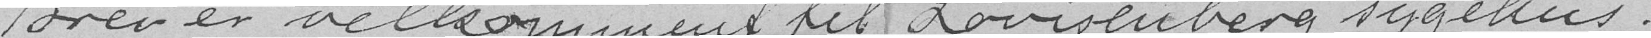Brev er velkomment til Lovisenberg sygehus.
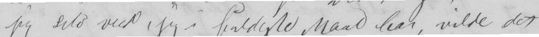jeg selv veed, jeg i fuldeste Maal har, vilde det
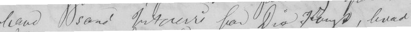have sand Interesse for Din Kunst, hvad
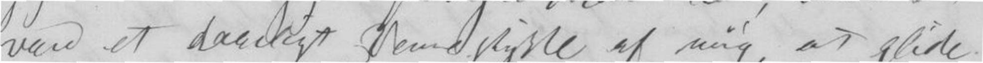være et daarligt Vennestykke af mig, at glide
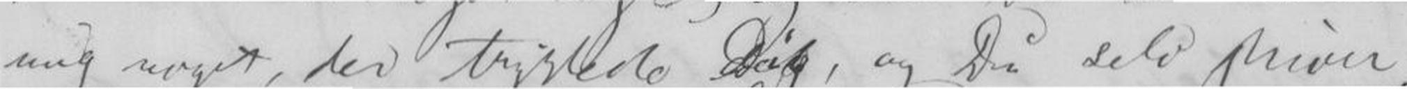mig noget, der trykkede Dig, og Du selv skriver,
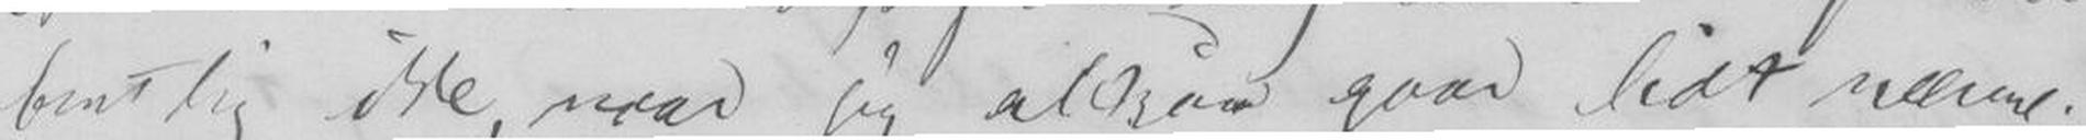bentlig ikke, naar jeg altsaa gaar lidt nærme-
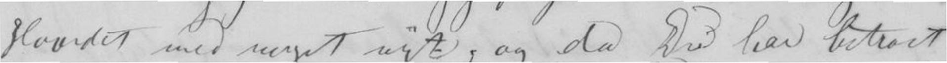Hovedet med meget nyt, og da Du har betroet
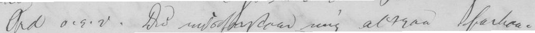Ord o.s.v. Du misforstaar mig altsaa forhaa-
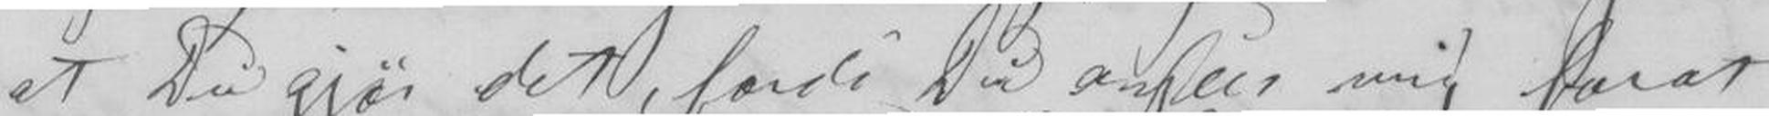at Du gjør det, fordi Du anseer mig forat
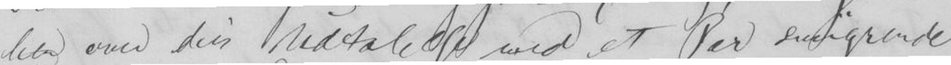hen over din Udtalelse med et Par smigrende
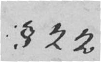§ 22.
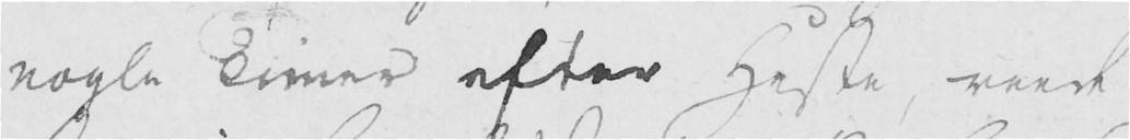nogle Timer efter Heste, reede
